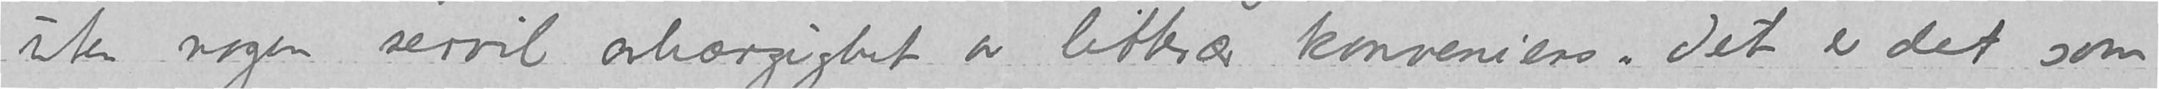uten nogen servil avhængighet av litterær konveniens. Det er det som
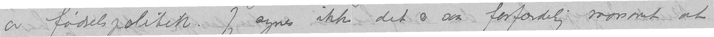av fødselspolitik. Jeg synes ikke det er saa forfærdelig morsomt at
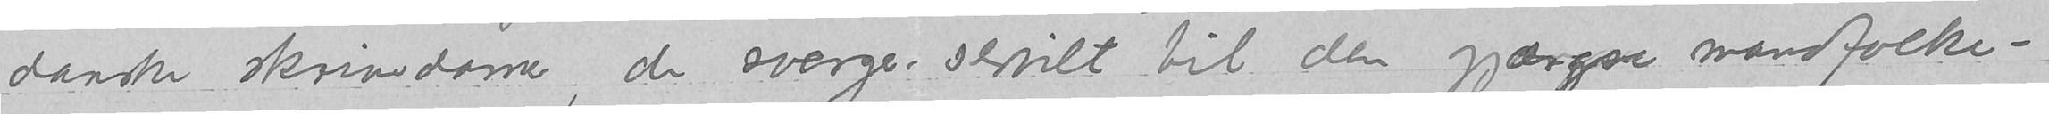danske skrivedamer, de sverger servilt til den gjængse mandfolk
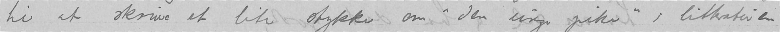til at skrive et lite stykke om «Den unge pike» i litteraturen
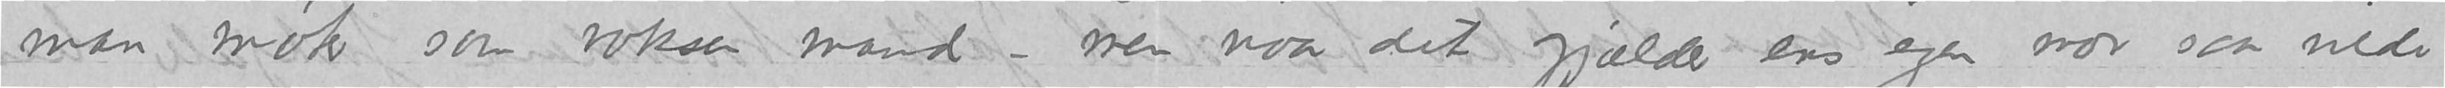man møter som voksen mand – men naar det gjælder ens egen mor saa vilde
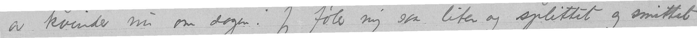av kvinder nu om dagen. Jeg føler mig saa liten og splittet og smittet
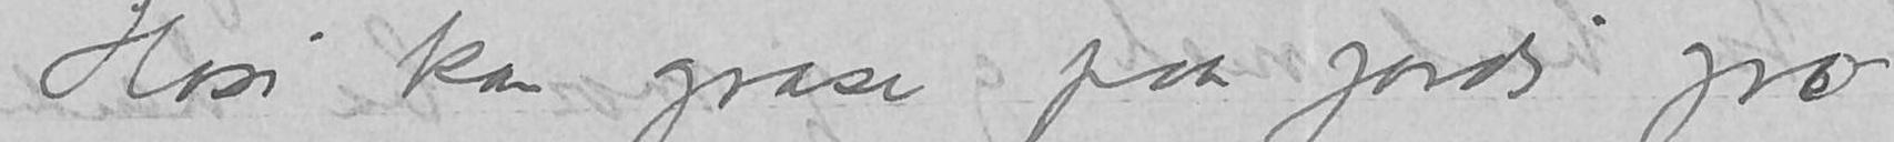Hosi kan grase paa jordi gro
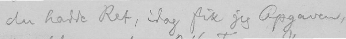du hadde Ret, idag fik jeg Opgaven,
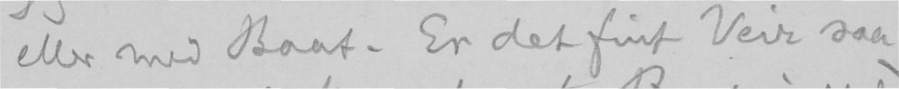eller med Baat. Er det fint Veir saa
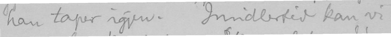han taper igjen. Imidlertid kan vi
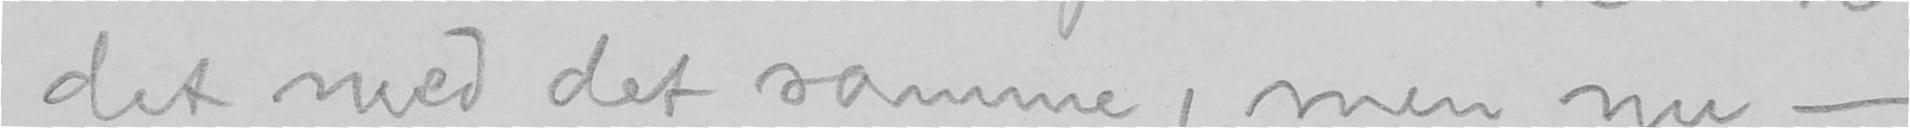det med det samme, men nu -
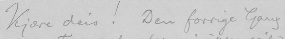Kjære deis! Den forrige Gang
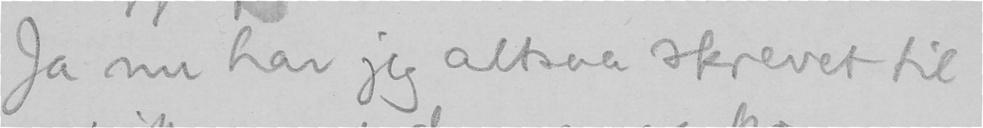Ja nu har jeg altsaa skrevet til
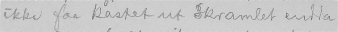ikke faa kastet ut Skramlet endda
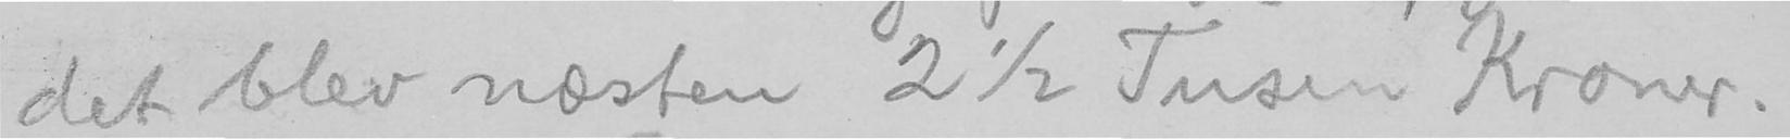det blev næsten 2 ½ Tusen Kroner.
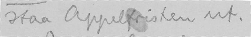staa Appelfristen ut.
